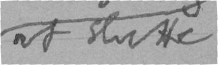at slutte
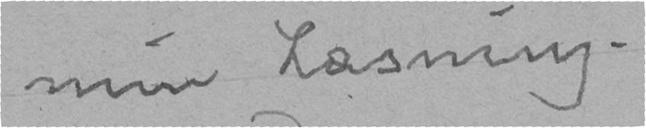min Læsning.
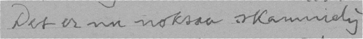Det er nu noksaa skammelig
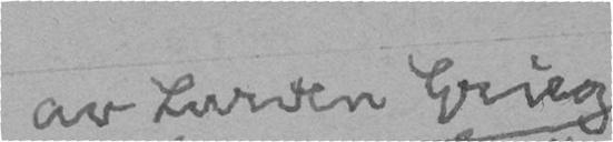av Lurven Grieg
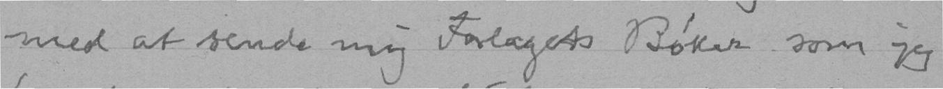med at sende mig Forlagets Bøker som jeg
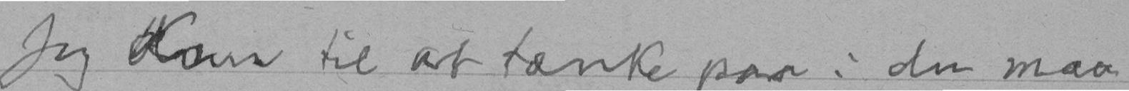Jeg kom til at tænke paa: du maa
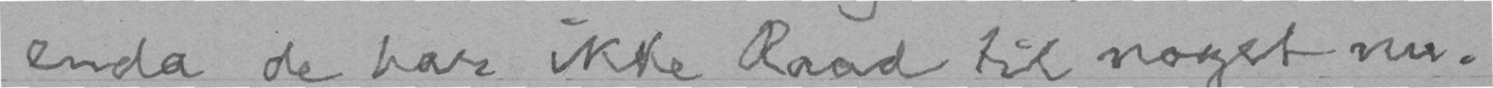enda de har ikke Raad til noget nu.
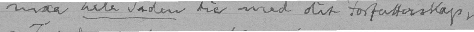maa hele Tiden tie med dit Forfatterskap,
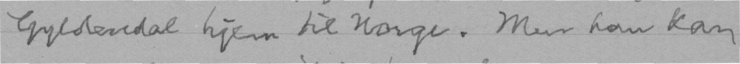Gyldendal hjem til Norge. Men han kan
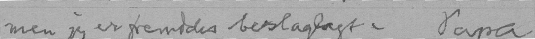men jeg er fremdeles beslaglagt. Papa
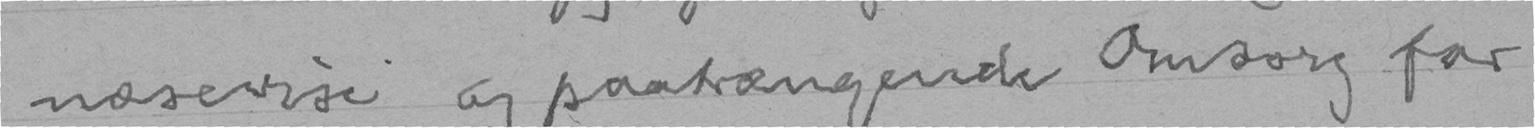næsevise og paatrængende Omsorg for
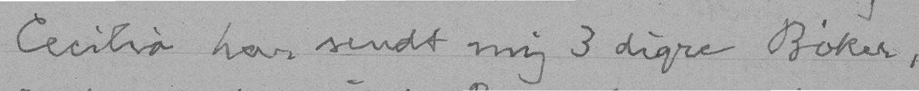Cecilia har sendt mig 3 digre Bøker,
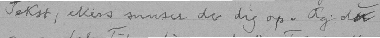Tekst, ellers snuser de dig op. Og du
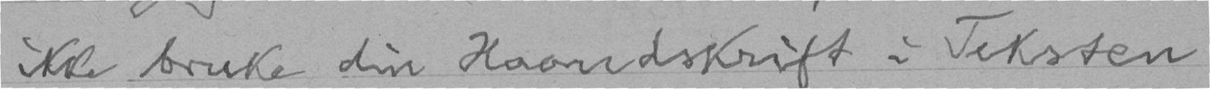ikke bruke din Haandskrift i Teksten
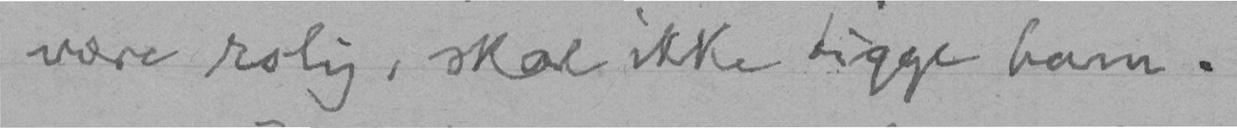være rolig, skal ikke tigge ham.
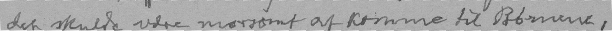det skulde være morsomt at komme til Børnene,
